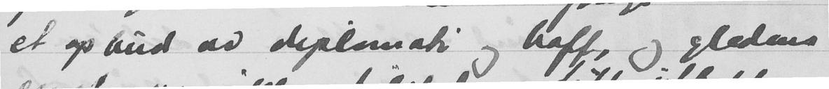et opbud av diplomati og hoff, og gledens
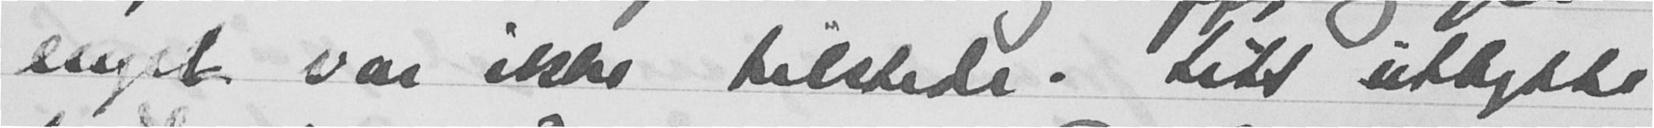engel var ikke tilstede. Litt utbytte
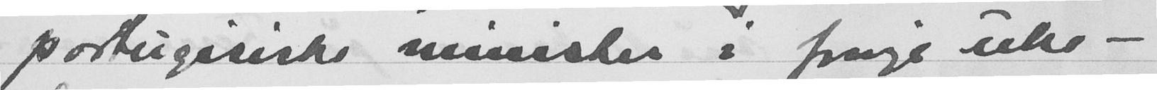portugisiske minister i forrge uke -
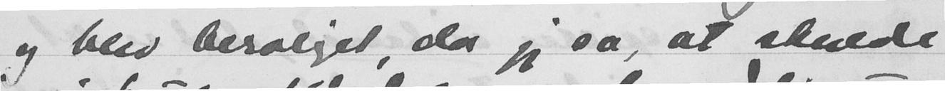og blev beroliget, da jeg sa, at skulde
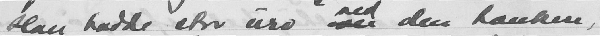Han hadde stor uro med den tanken,
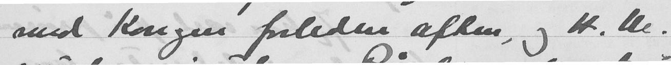med Kongen forleden aften, og H. M.
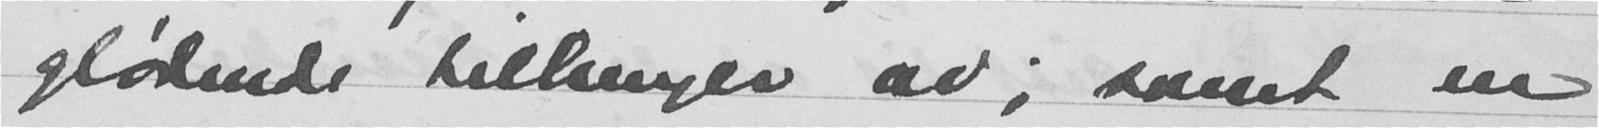glødende tilhenger av; samt en
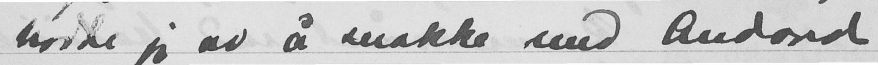hadde jeg av å snakke med Andland
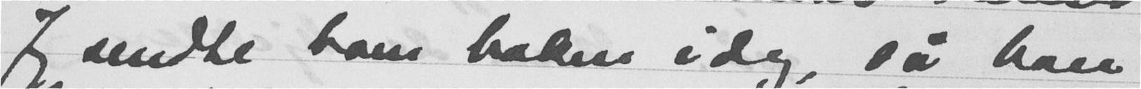Jeg sendte ham boken idag, så han
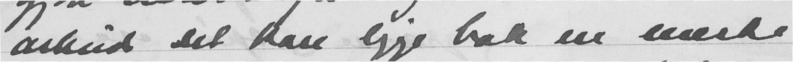arbeid det kan ligge bak en eneste
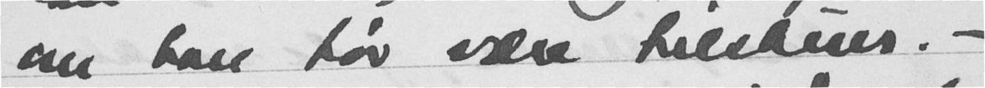om han tør være tilskuer. -
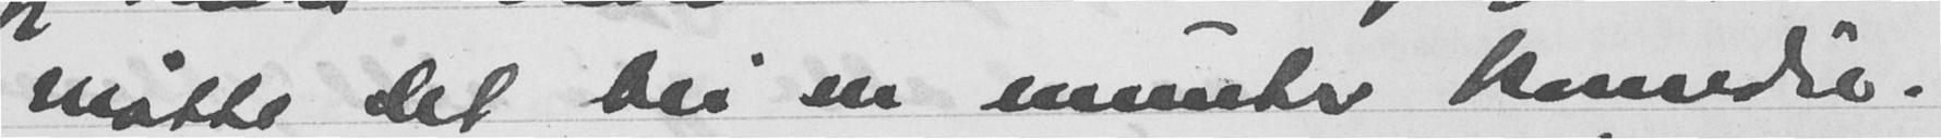måtte det bli en munter komedie.
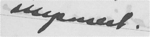imponert.
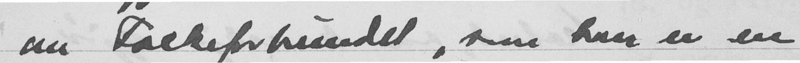om Folkeforbundet, som han er en
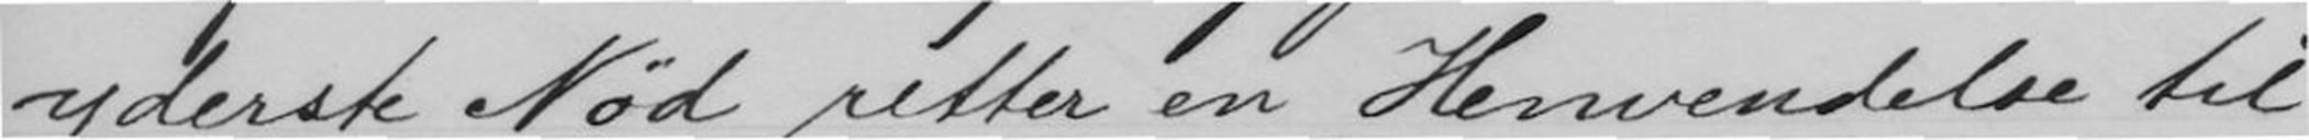yderste Nød retter en Henvendelse til
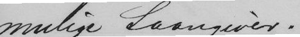mulige Laangiver.
